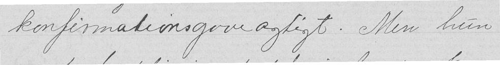konfirmationsgaveagtigt. Men hun
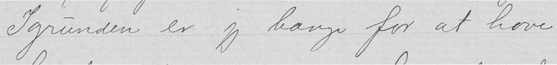Igrunden er jeg bange for at have
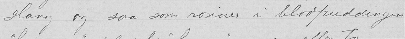slang og saa som rosiner i blodpuddingen
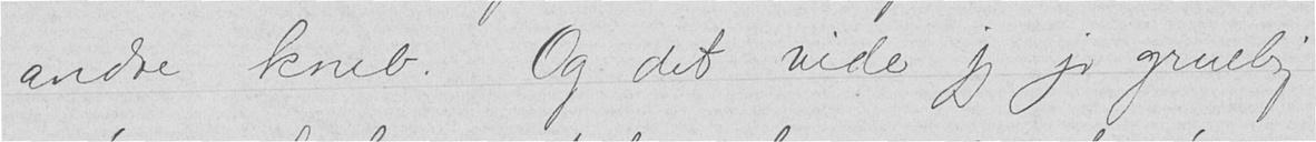andre kneb. Og det vilde jeg jo gruelig
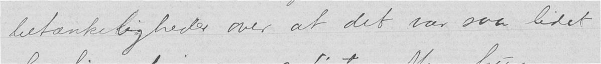betænkeligheder over at det var saa lidet
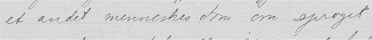et andet menneskes dom om sproget
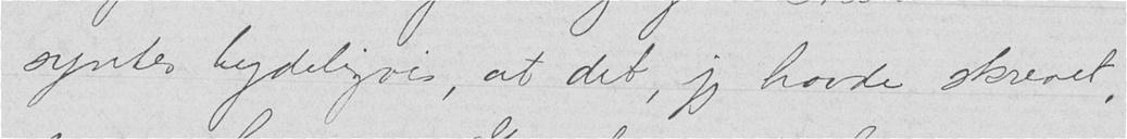syntes tydeligvis, at det, jeg havde skrevet,
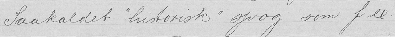Saakaldet "historisk" sprog som f.ex.
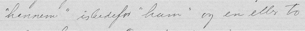"hannem" istedenfor "ham" og en eller to
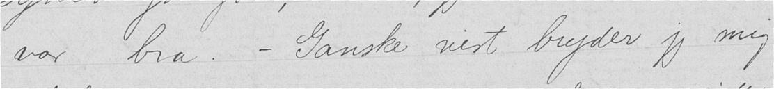var bra. - Ganske vist bryder jeg mig
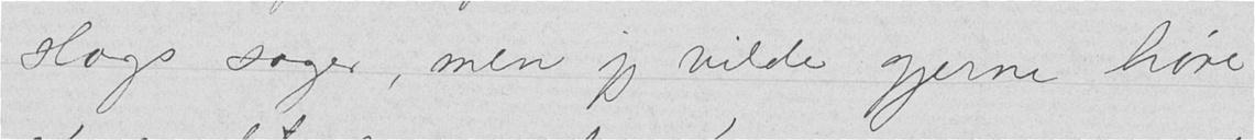slags sager, men jeg vilde gjerne høre
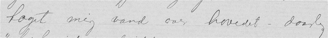taget meg vand over hovedet - daarlig
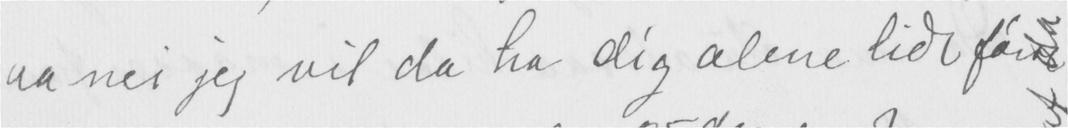aa nei jeg vil da ha dig alene lidt først
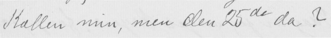Kallen min, men den 25de da?
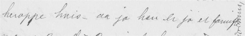heroppe hvis – aa jo han er jo et familie-
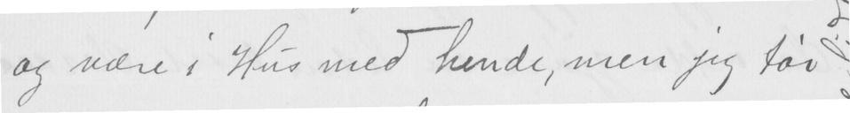og være i Hus med hende, men jeg tør
